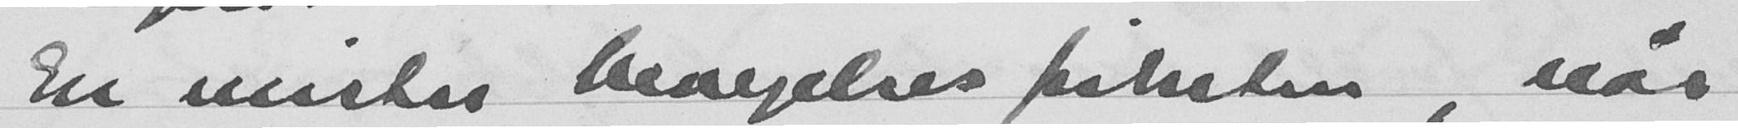En mister bevegelses friheten, når
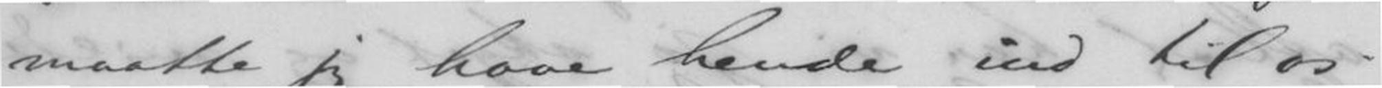maatte jeg have hende ind til os
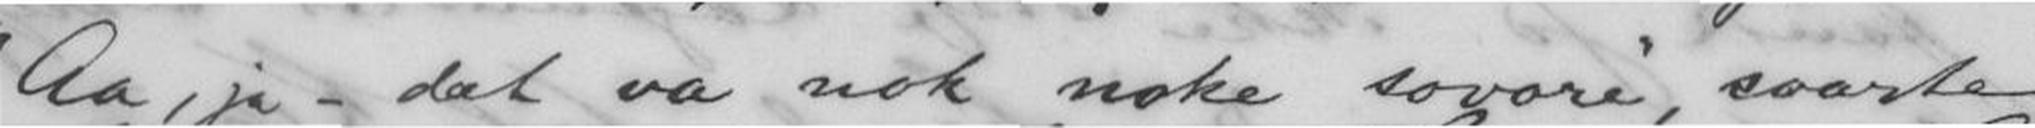"Aa, ja - det va nok noke sovore", svarte
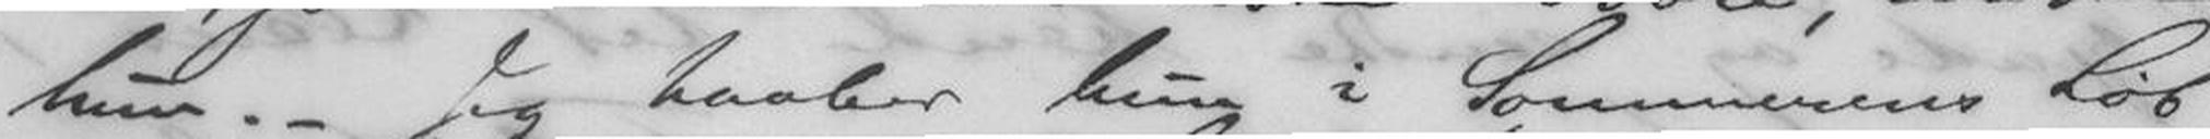hun. - Jeg haaber hun i Sommerens Løb
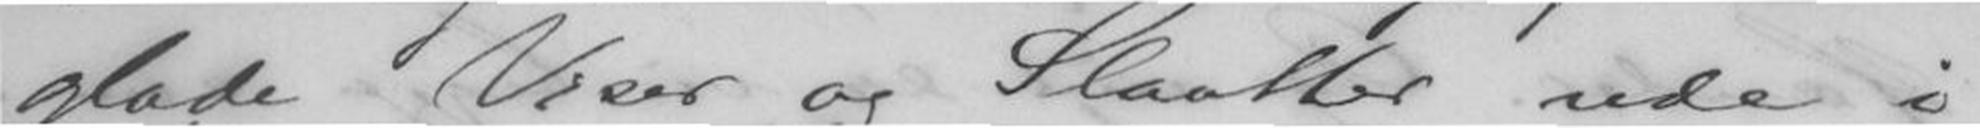glade Viser og Slaatter ude i
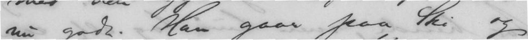nu godt. Han gaar paa Ski og
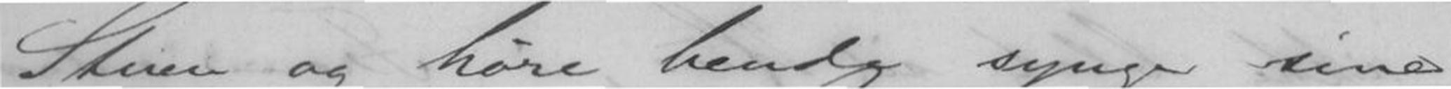Stuen og høre hende synge sine
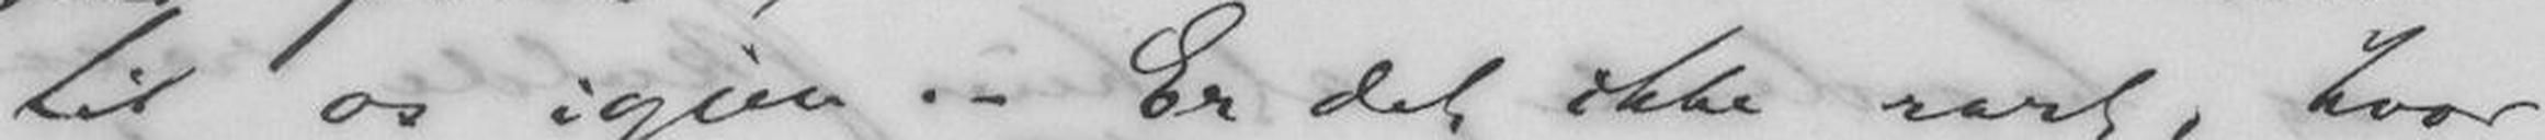til os igjen. - Er det ikke rart, hvor
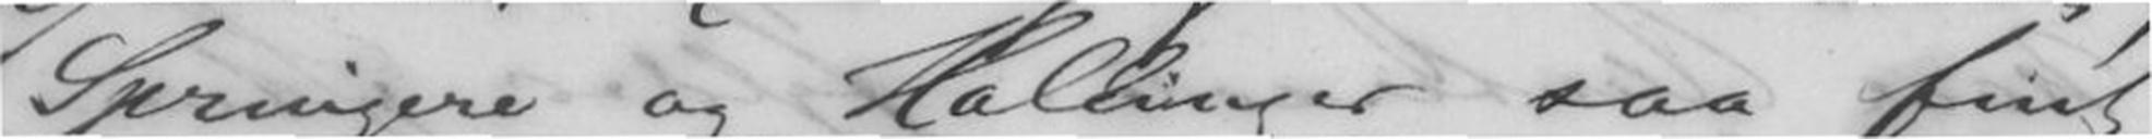Springere og Hallinger saa fint
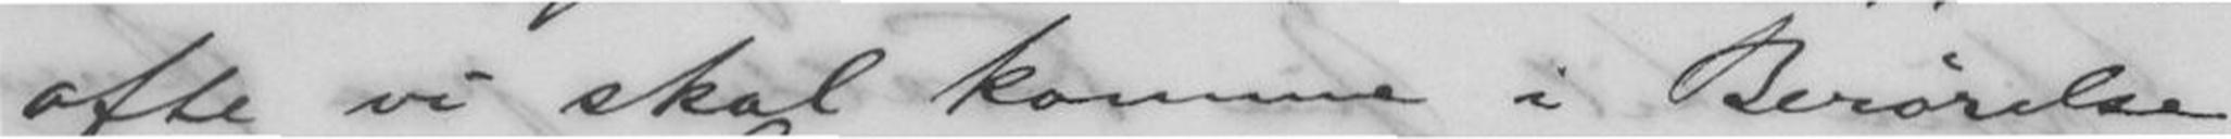ofte vi skal komme i Berørelse
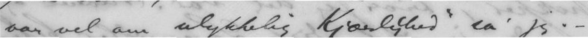var vel om ulykkelig Kjærlighed" sa jeg. -
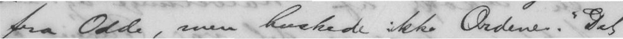fra Odda, men huskede ikke Ordene. "Det
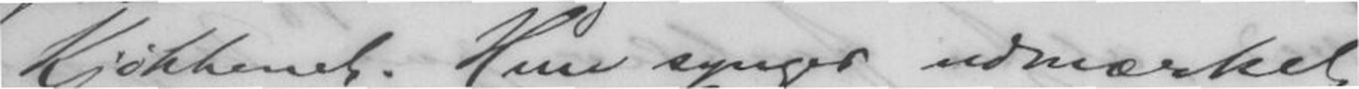Kjøkkenet. Hun synger udmærket,
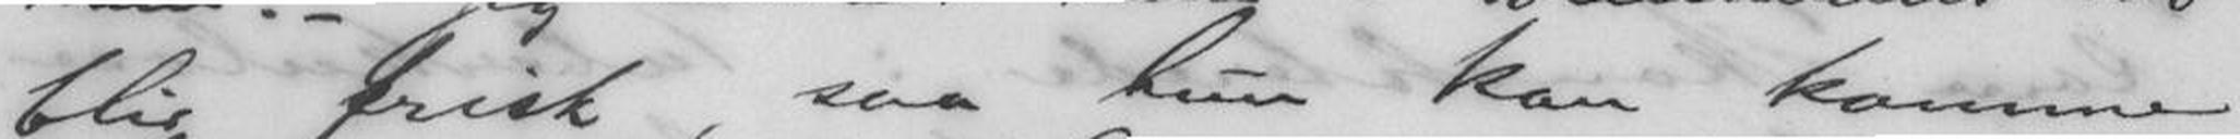blir frisk, saa hun kan komme
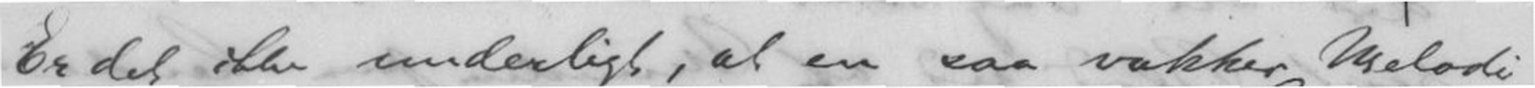Er det ikke underligt, at en saa vakker Melodi
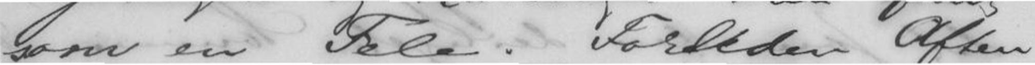som en Fele. Forleden Aften
